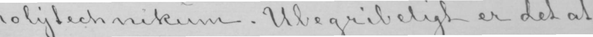polytechnikum. Ubegribeligt er det at
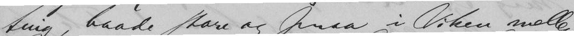ting, baade store og smaa i Viken mellem
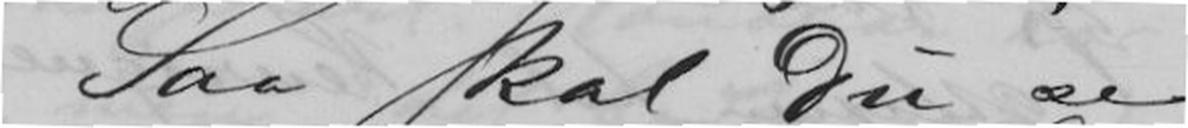Saa skal Du se
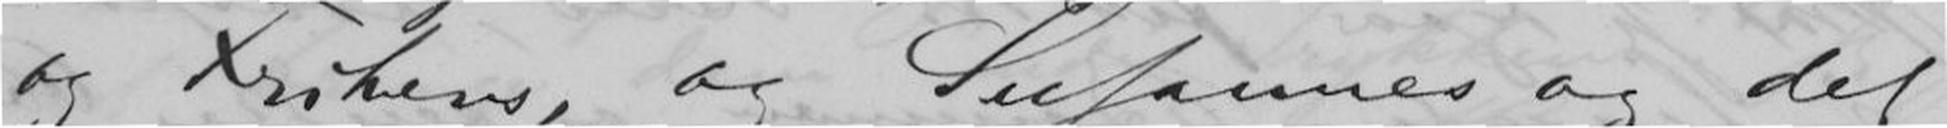og Frikens, og Susannes og det
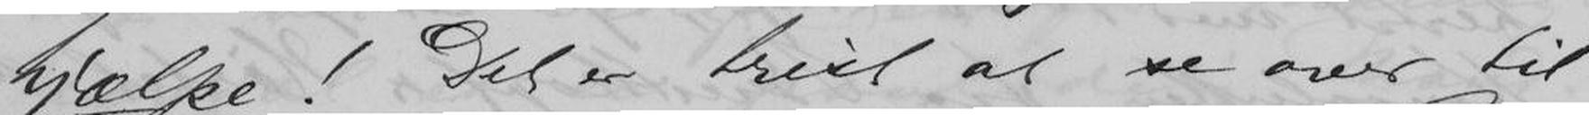hjælpe! Det er trist at se over til
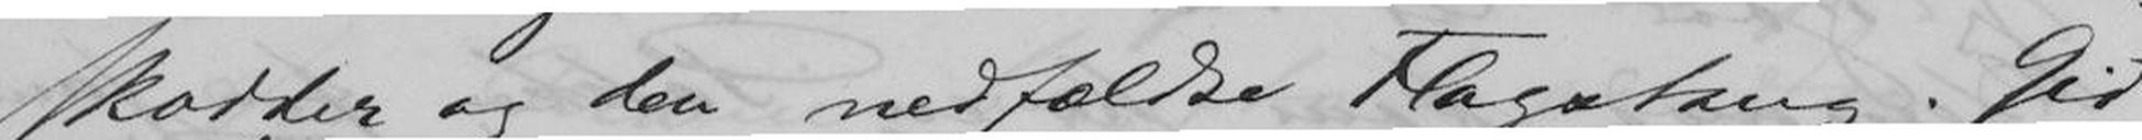skodder og den nedfældte Flagstang. Gid
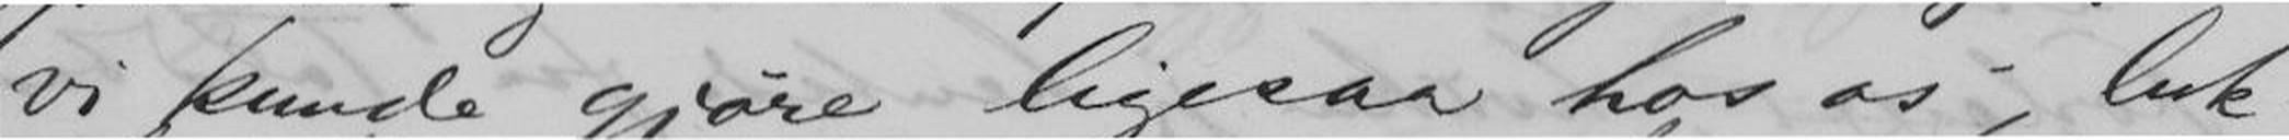vi kunde gjøre ligesaa hos os; luk-
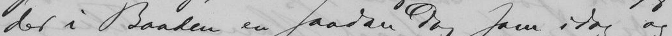der i Baaden en saadan Dag som idag og
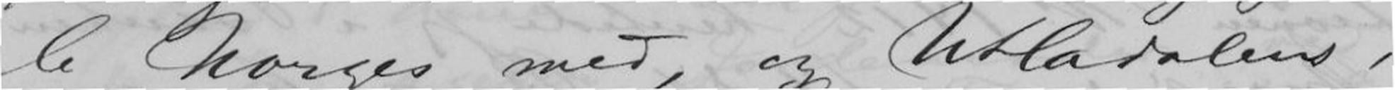le Norges med, og Utladalens,
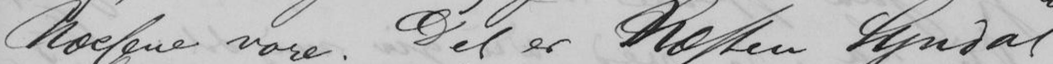Næssene vore. Det er Næsten Synd at
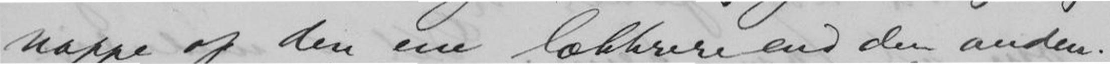nappe op den ene lækkrere end den anden.
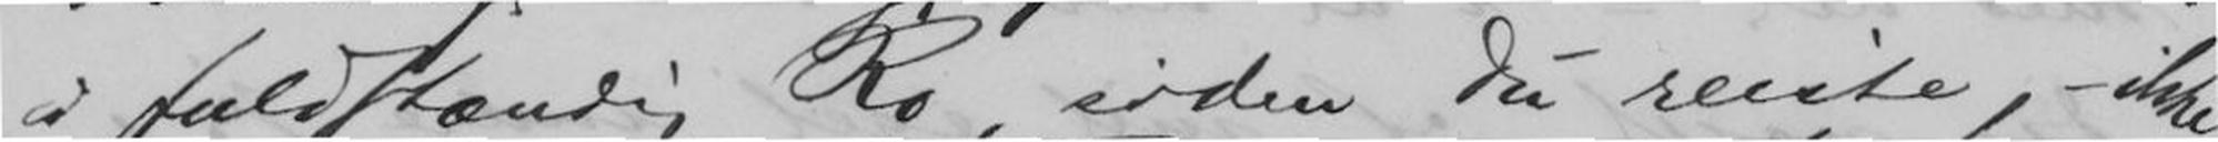i fuldstændig Ro, siden du reiste, - ikke
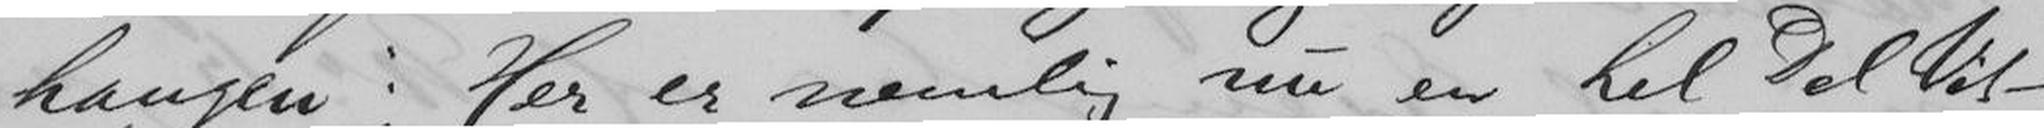haugen: Her er nemlig nu en hel Del Vit-
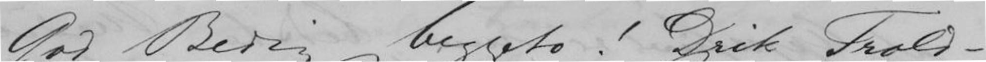God Bedring beggeto! Drik Trold-
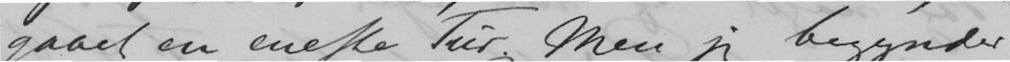gaaet en eneste Tur. Men jeg begynder
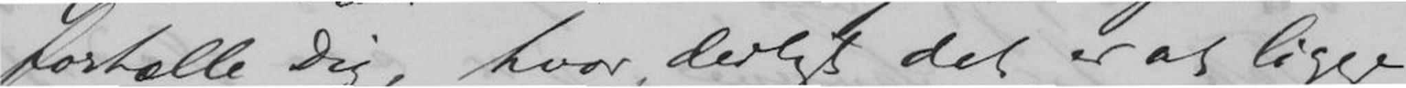fortælle dig, hvor deiligt det er at ligge
